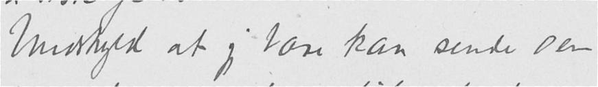Undskyld at jeg bare kan sende Dem
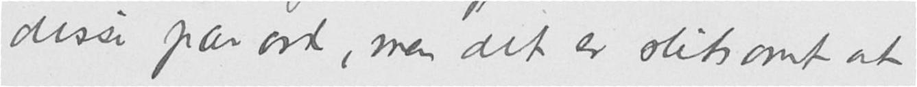disse par ord, men det er slitsomt at
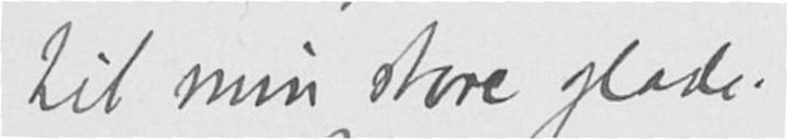til min store glæde.
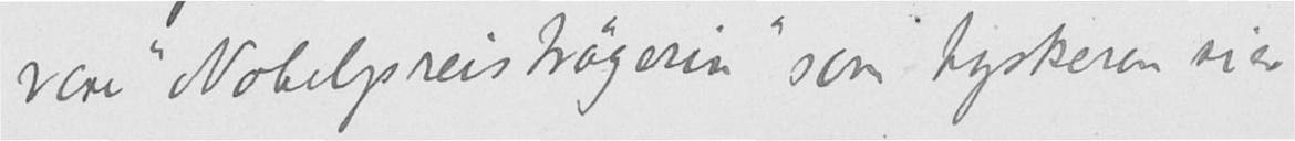være "Nobelpreisträgerin" som tyskeren sier
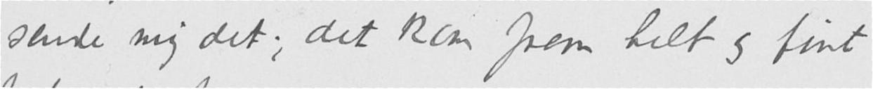sende mig det; det kom frem helt og fint
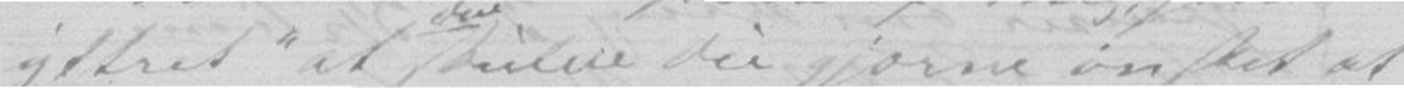yttret at den skulde du gjærne ønsket at
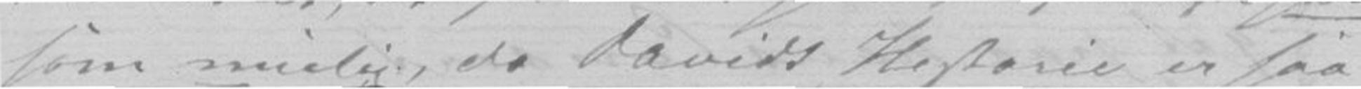som mulig, da Davids Historie er saa
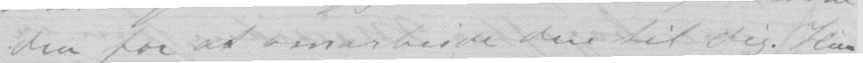den for at omarbeide den til dig. Hun
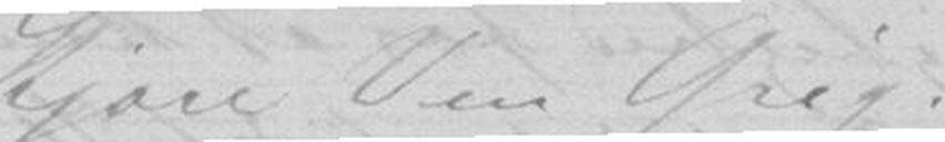Kjære Ven Grig!
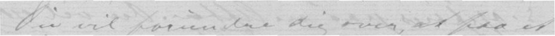Du vil forundre dig over, at faa et
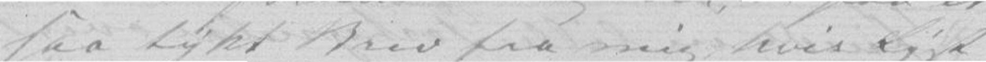saa tykt Brev fra mig hvis Lyst
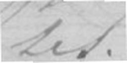tet.
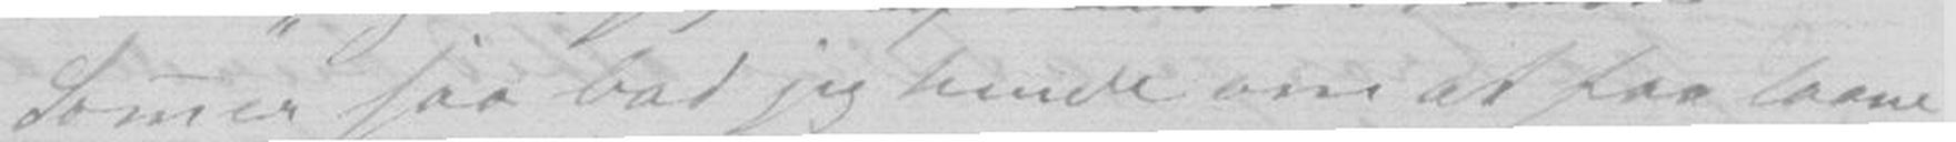Sommer saa bad jeg hende om at faa laane
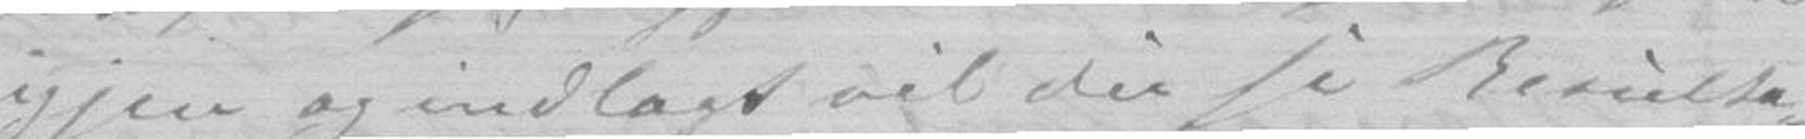igjen og indlagt vil du se Resulta-
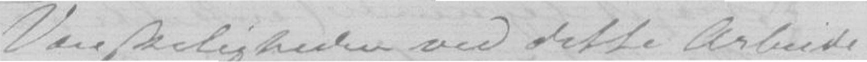Vanskeligheden ved dette Arbeide
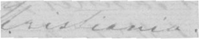Kristiania.
